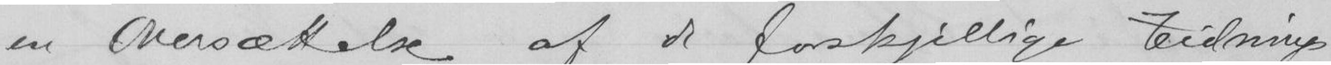en Oversættelse af de forskjellige tidnings
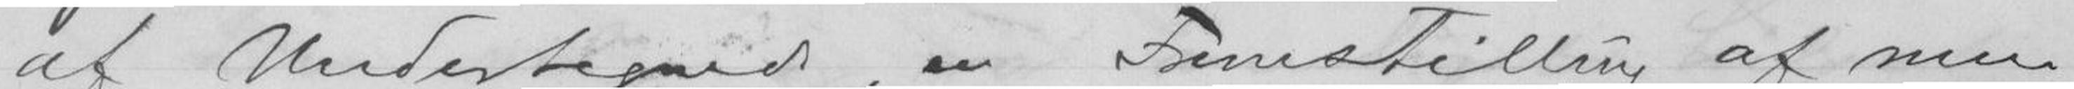af Undertegnede, en Fremstilling af mus-
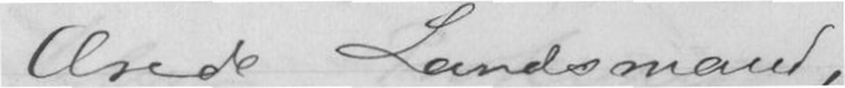Ærede Landsmand,
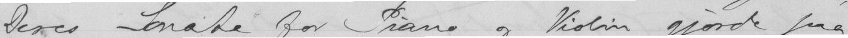Deres Sonate for Piano og Violin gjorde paa
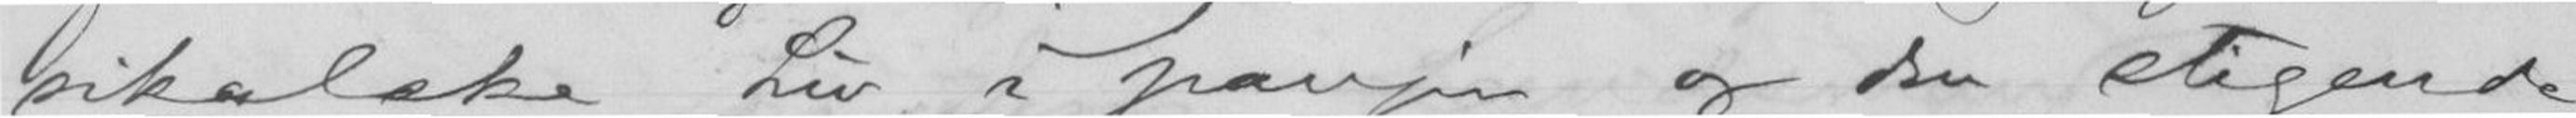ikalske Liv i Spanjen og den stigende
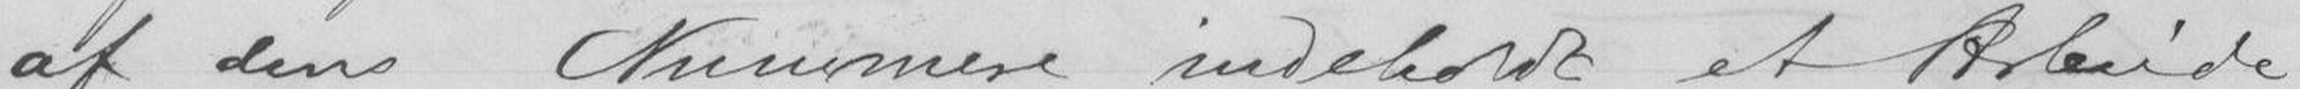af dens Nummere indeholdt et Arbeide
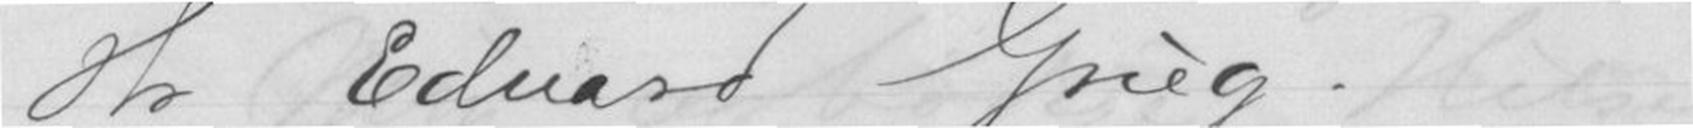Hr Edvard Grieg.
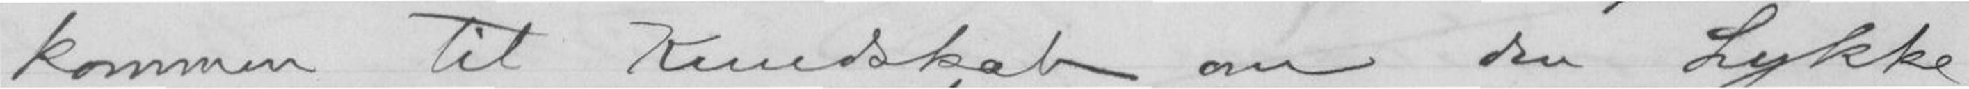kommen til Kundskab om den Lykke,
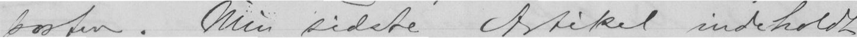posten. Min sidste Artikel indeholdt
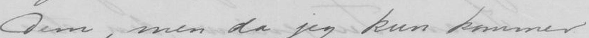Dem, men da jeg kun kommer
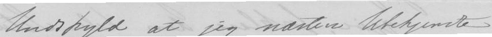Undskyld at jeg næsten Ubekjendte
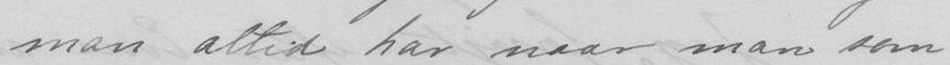man altid har naar man som
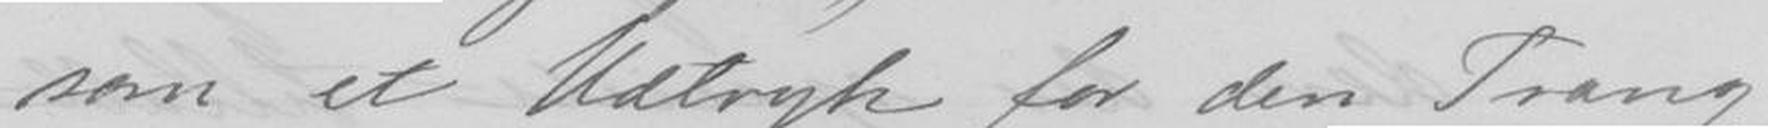som et Udtryk for den Trang
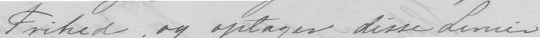Frihed og optager disse Linier
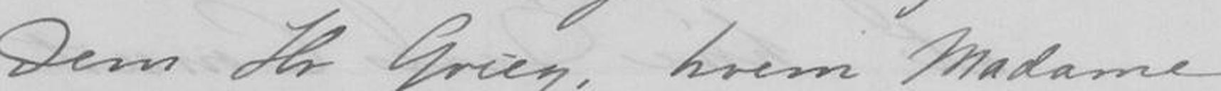Dem Hr Grieg, hvem Madame
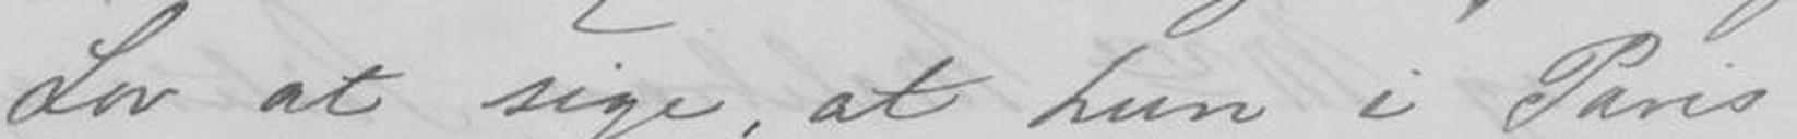Lov at sige, at hun i Paris
